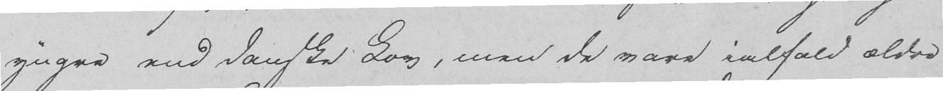yngre end danske Lov, men de vare ialfald ældre
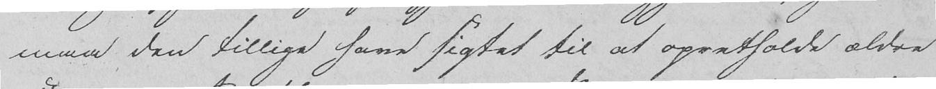maa den tillige have sigtet til at opretholde ældre
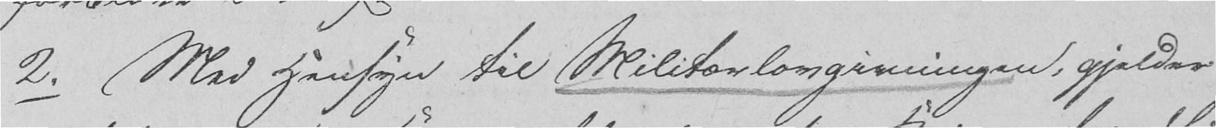2. Med Hensyn til Militærlovgivningen, gjelder
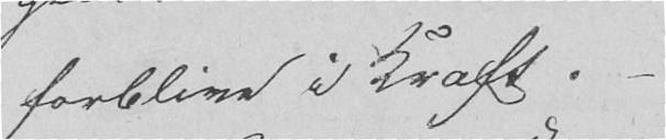forblive i Kraft. -
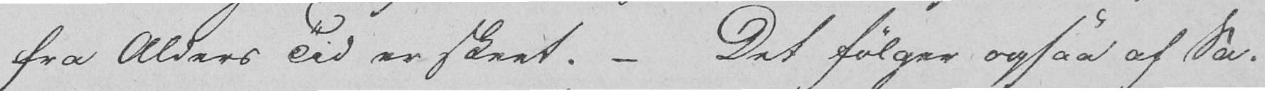fra Alders Tid er skeet. - Det følger ogsaa af Sa-
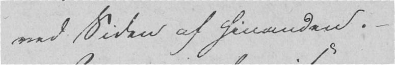ved Siden af hinanden. -
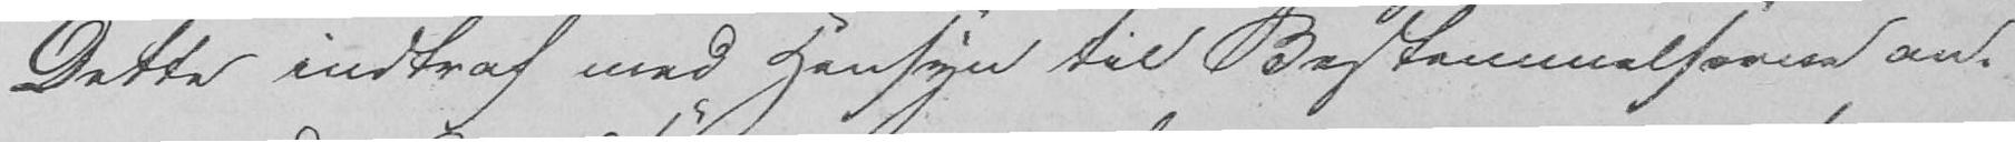Dette indtraf med Hensyn til Bestemmelserne an-
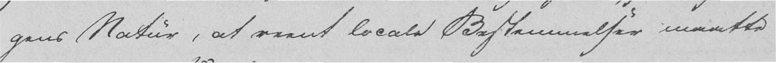gens Natur, at reent locale Bestemmelser maatte
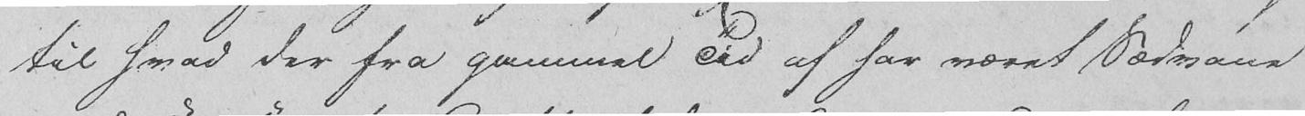til hvad der fra gammel Tid af har været Sædvane
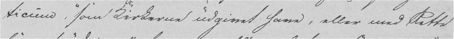ticum, "som Kirkerne udgivet have, eller med Rette
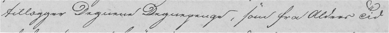tillægger Degnene Degnepenge, som fra Alders Tid
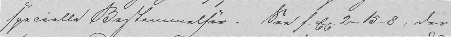specielle Bestemmelser. See f. Ex. 2-15-8, der
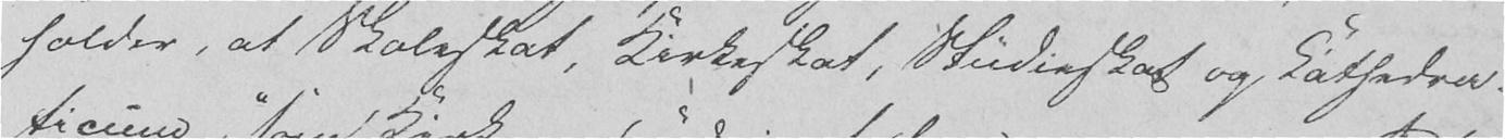holder, at Skoleskat, Kirkeskat, Studieskat og Cathedra-
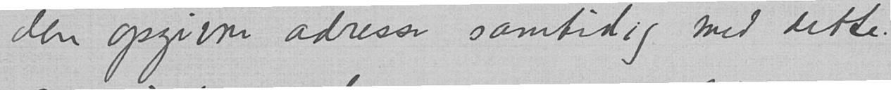den opgivne adresse samtidig med dette.
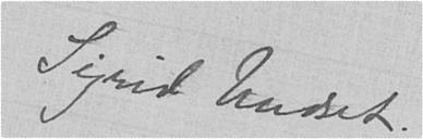Sigrid Undset.
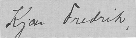Kjære Fredrik,
